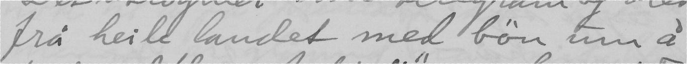frå heile landet med bön um å
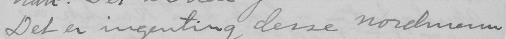Det er ingenting desse nordmenn
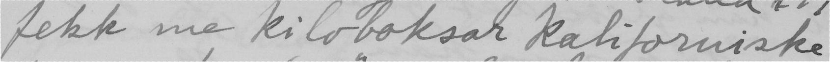fekk me kiloboksar kaliforniske
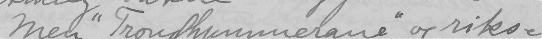Men "Trondhjemmerane" og riks-
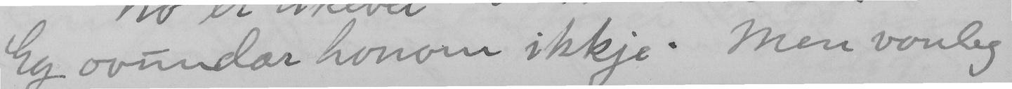Eg ovundar honom ikkje. Men vonleg
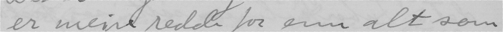er meire redde for enn alt som
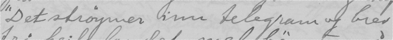"Det ströymer inn telegram og brev
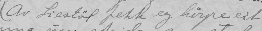Av Liestöl fekk eg höyre eit
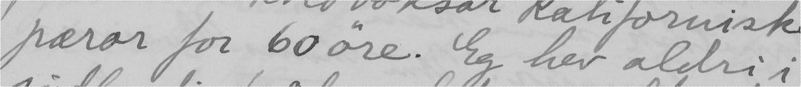pærar for 60 öre. Eg hev aldri i
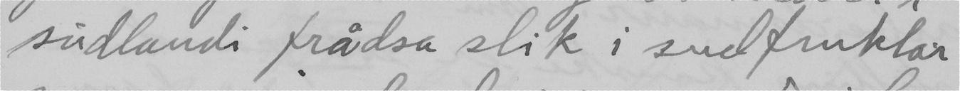sudlandi frådsa slik i sudfruktar
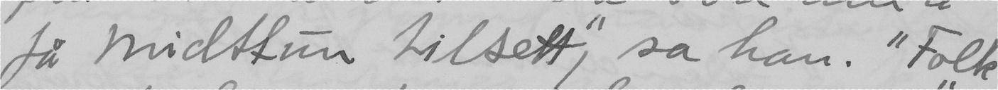få midttun tilsett," sa han. "Folk
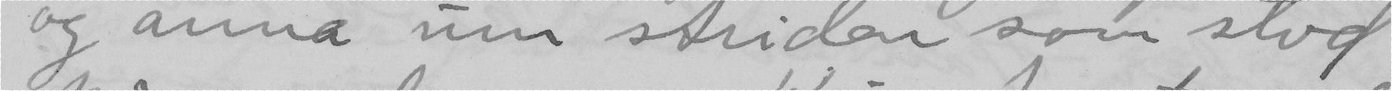og anna um striden som stod
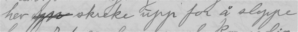hev skrike upp for å slippe
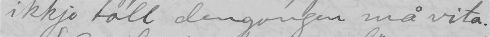ikkje toll dengangen må vita.
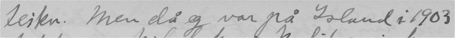teikn. Men då eg var på Island i 1903
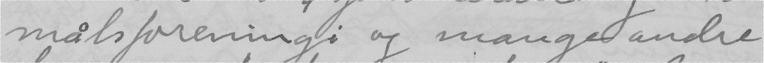målsforeningi og mange andre
